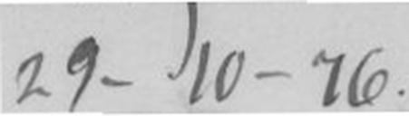29-10-76.
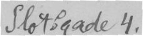Slotsgade 4.
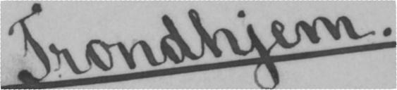Trondhjem.
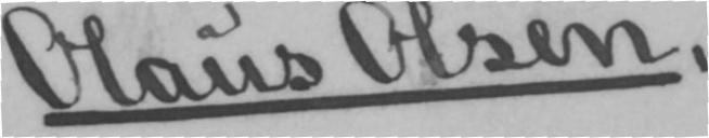Olaus Olsen,
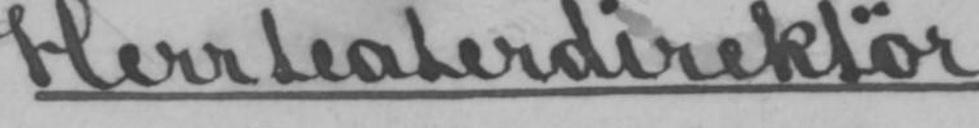Herr teaterdirektør
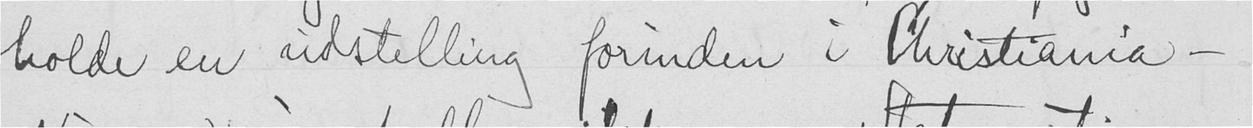holde en udstilling forinden i Christiania -
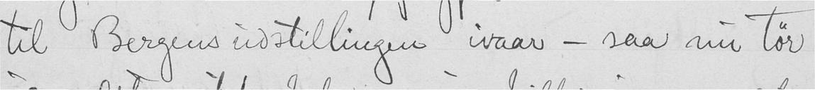til Bergens udstillingen ivaar, - saa nu tør
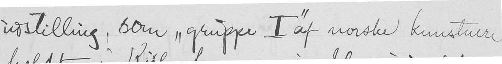udtilling, som "gruppe 1" af norske kunstnere
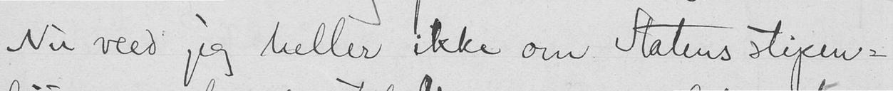Nu veed jeg heller ikke om Statens stipen-
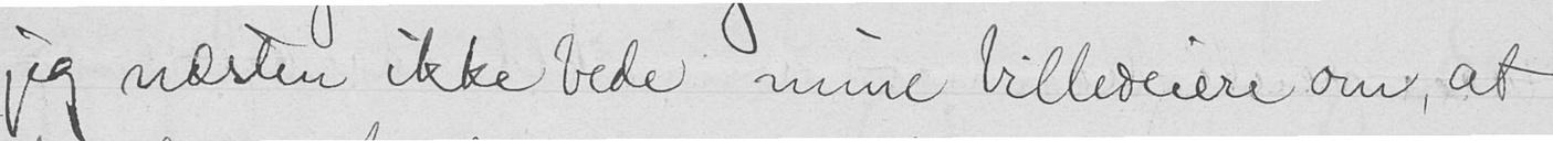jeg næsten ikke bede mine billedeiere om, at
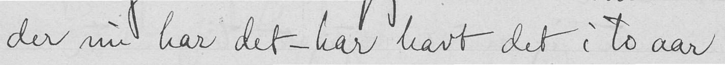der nu har det - har havt det i to aar
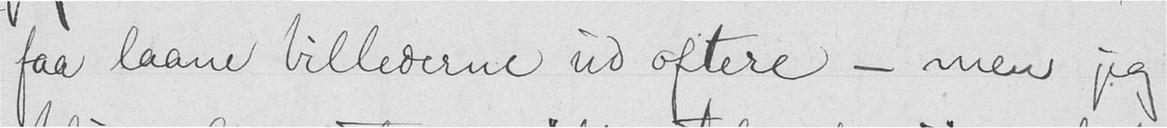faa laane billederne ud oftere - men jeg
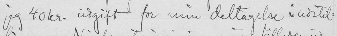jeg 40 kr. udgift for min deltagelse i udstil-
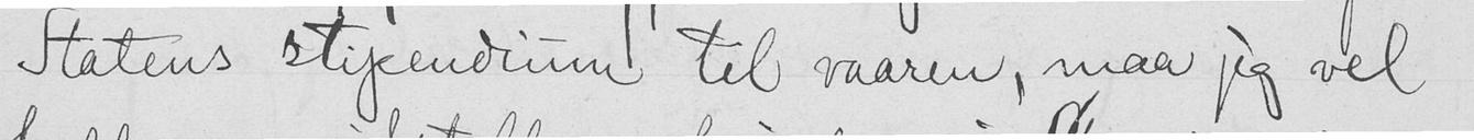Statens stipendium til vaaren, maa jeg vel
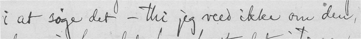i at søge det - thi jeg veed ikke om den,
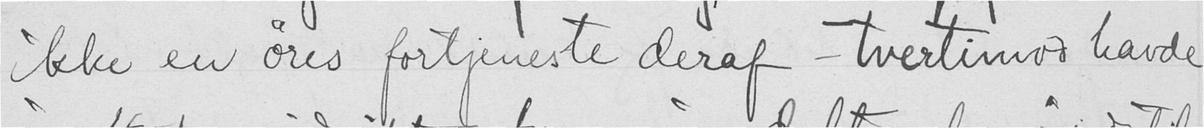ikke en øres fortjeneste deraf - tvertimod havde
